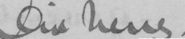Din heng.
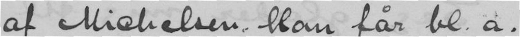af Michelsen. Han får bl.a.
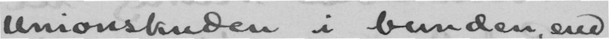unionskuden i bunden, end
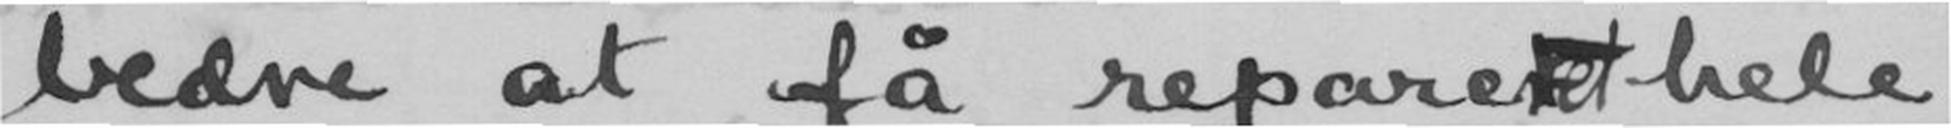bedre at få repareret hele
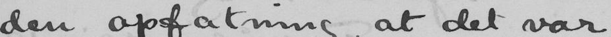den opfatning, at det var
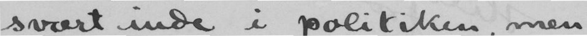svært inde i politiken, men
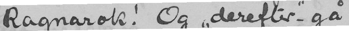Ragnarok! Og derefter - gå
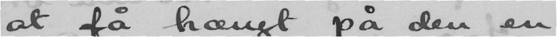at få hængt på den en
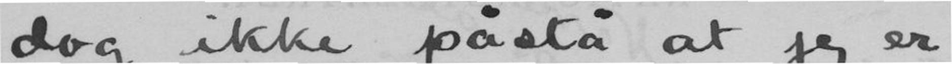dog ikke påstå at jeg er
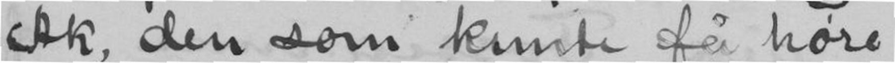Ak, den som kunde få høre
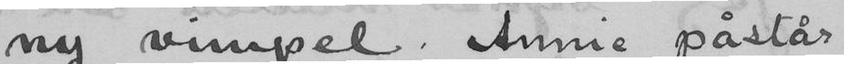ny vimpel. Annie påstår
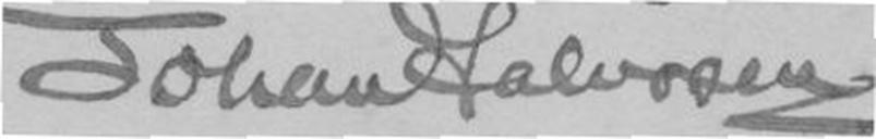Johan Halvorsen
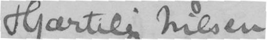Hjærtelig hilsen
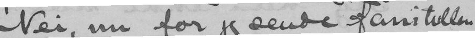Nei, nu for jeg sende Fanitullen
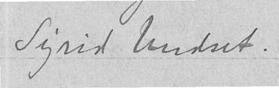Sigrid Undset.
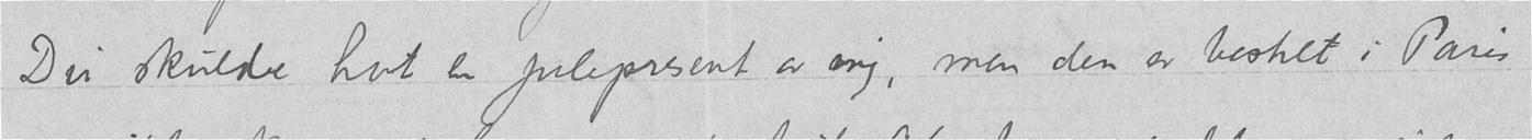Du skulde hat en julepresent av mig, men den er bestilt i Paris
